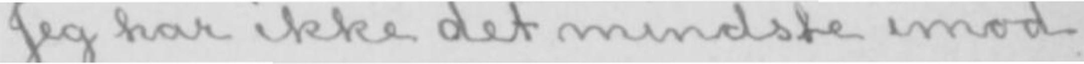Jeg har ikke det mindste imod
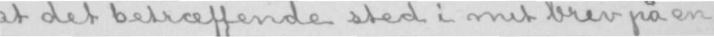at det betræffende sted i mit brev på en
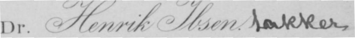Dr. Henrik Ibsen. takker
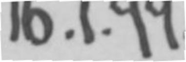16.1.99.
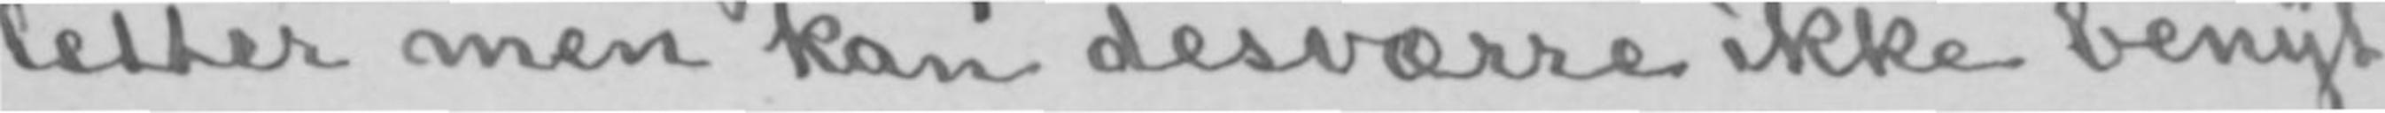letter men kan desværre ikke benyt-
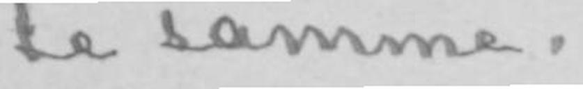te samme.
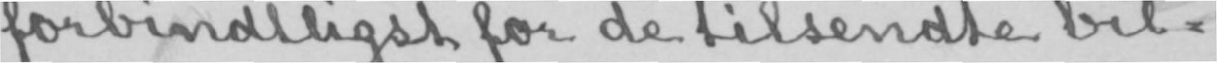forbindtligst for de tilsendte bil-
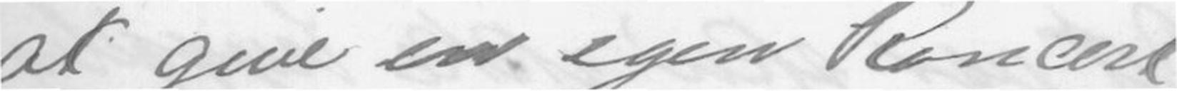at give en egen koncert
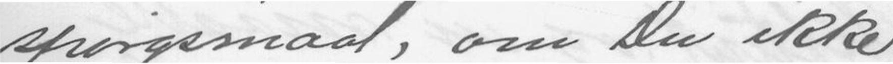spørgsmaal, om Du ikke
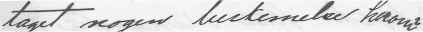taget nogen bestemelse herom?
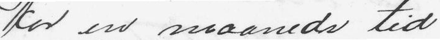For en maaneds tid
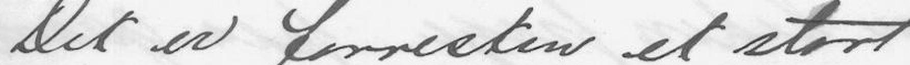Det er forresten et stort
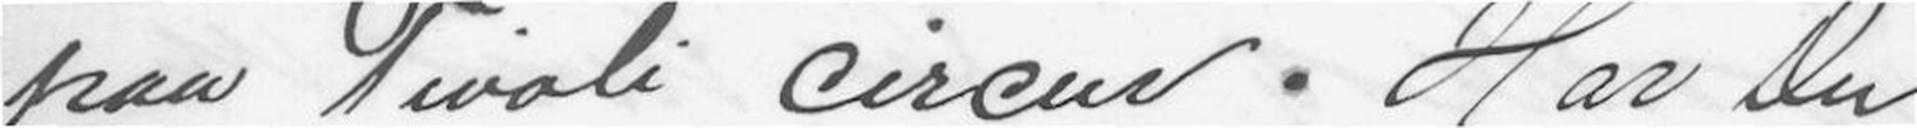paa Tivoli circus. Har Du
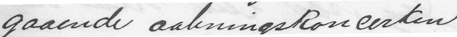gaaende aabningskoncerten
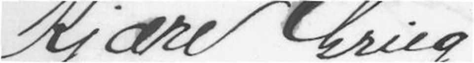Kjære Grieg
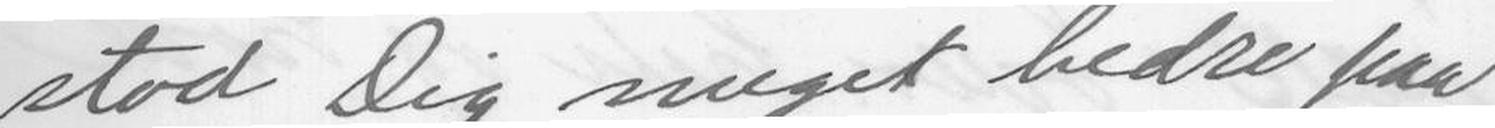stod Dig meget bedre paa
